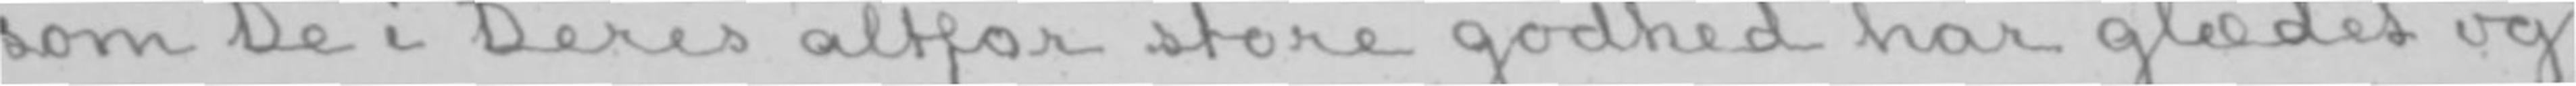som De i Deres altfor store godhed har glædet og
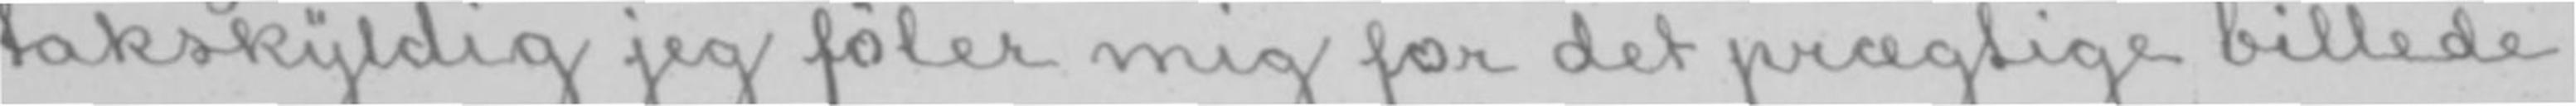takskyldig jeg føler mig for det prægtige billede,
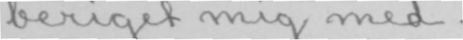beriget mig med.
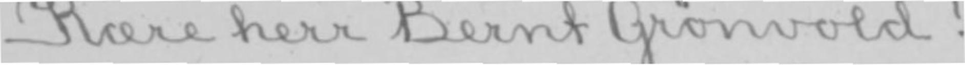Kære herr Bernt Grønvold!
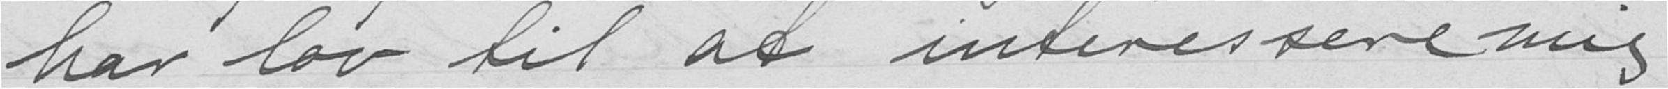har lov til at interessere mig
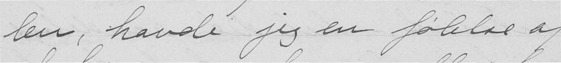len, havde jeg en følelse af
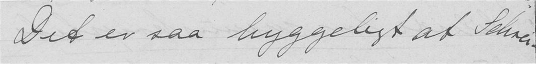Det er saa hyggeligt at Schrei-
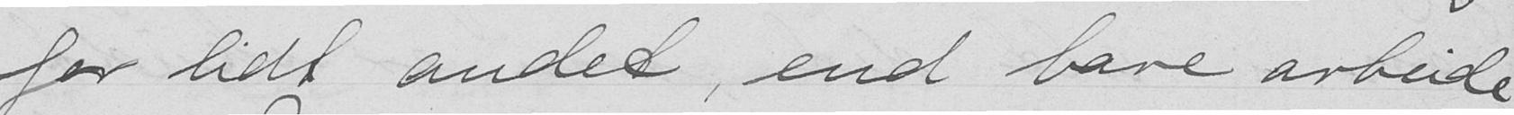for lidt andet, end bare arbeide.
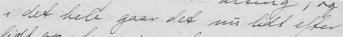i det hele gaar det nu lidt efter
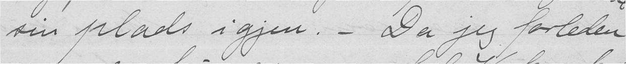sin plads igjen. - Da jeg forleden
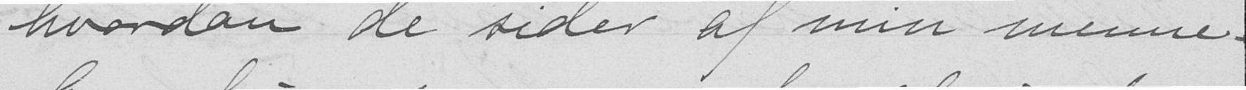hvordan de sider af min menne-
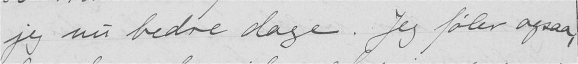jeg nu bedre dage. Jeg føler ogsaa,
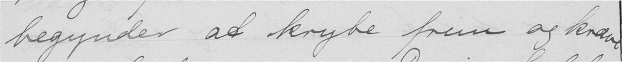begynder at krybe frem og kræve
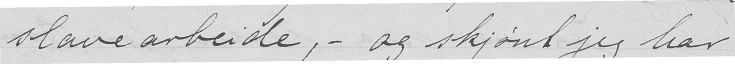slavearbeide, - og skjønt jeg har
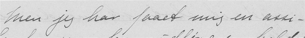Men jeg har faaet mig en assi-
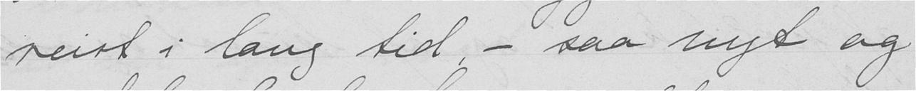reist i lang tid, - saa nyt og
